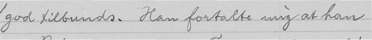god tilbunds. Han fortalte mig at han
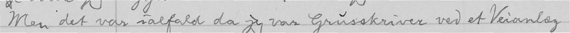Men det var ialfald da jeg var Grusskriver ved et Veianlæg
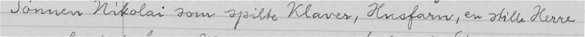Sønnen Nikolai som spilte Klaver, Husfarn, en stille Herre
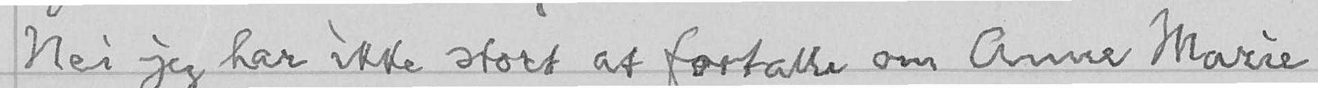Nei jeg har ikke stort at fortælle om Anne Marie
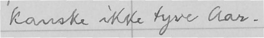kanske ikke tyve Aar.
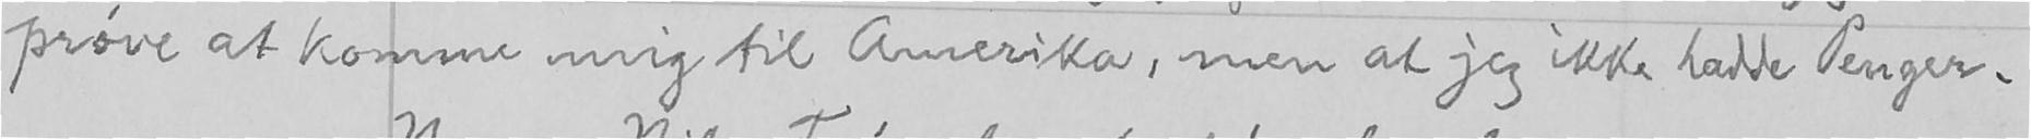prøve at komme mig til Amerika, men at jeg ikke hadde Penger.
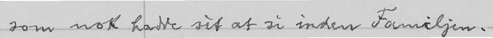som nok hadde sit at si inden Familjen.
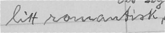litt romantisk,
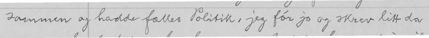sammen og hadde fælles Politik, jeg før jo og skrev litt da
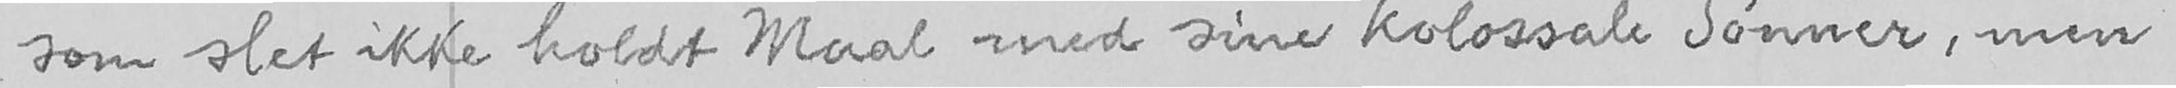som slet ikke holdt Maal med sine kolossale Sønner, men
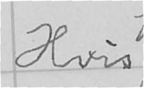Hvis
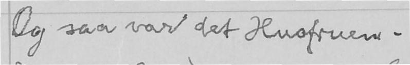Og saa var det Husfruen.
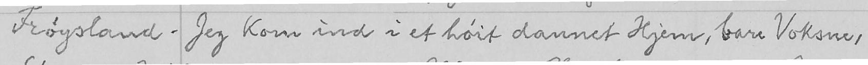Frøysland. Jeg kom ind i et høit dannet Hjem, bare Voksne,
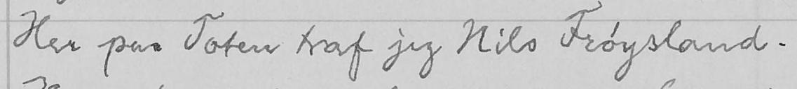Her paa Toten traf jeg Nils Frøysland.
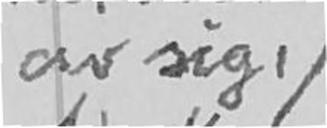av sig,
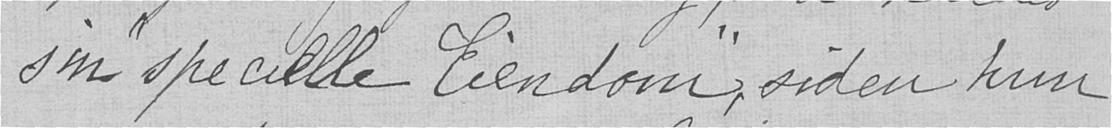sin "specielle Eiendom", siden hun
